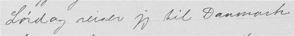Lørdag reiser jeg til Danmark
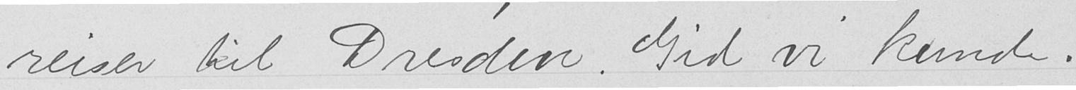reiser til Dresden. Gid vi kunde!
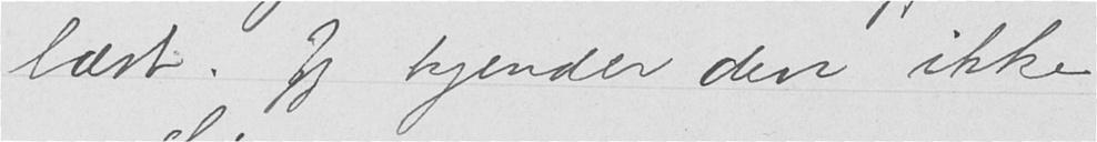læst. Jeg kjender den ikke.
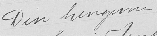Din hengivne
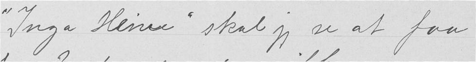"Inga Heine" skal jeg se at faa
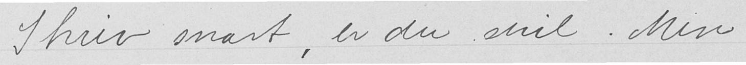Skriv snart, er du snil. Men
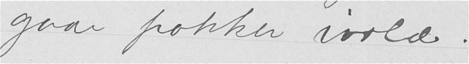gaar pokker ivold.
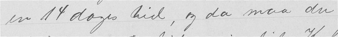en 14 dages tid, og da maa du
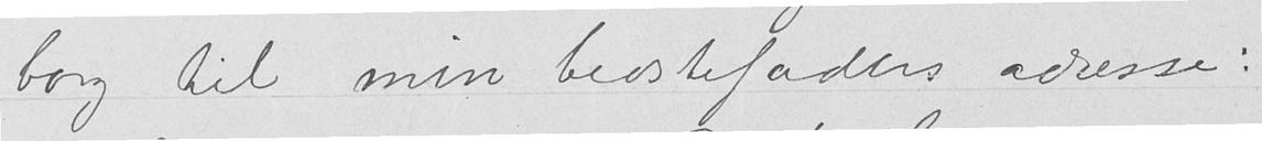borg til min bedstefaders adresse:
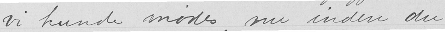vi kunde mødes, nu inden du
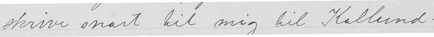skrive snart til mig til Kallund-
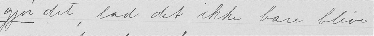gjør det, lad det ikke bare blive
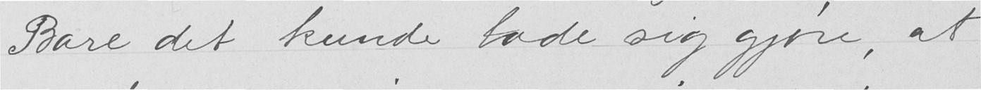Bare det kunde lade sig gjøre, at
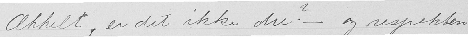ækkelt, er det ikke du? - og respekten
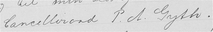Cancelliraad P. A. Gyth.
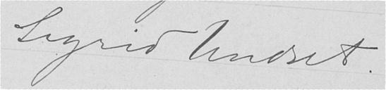Sigrid Undset.
